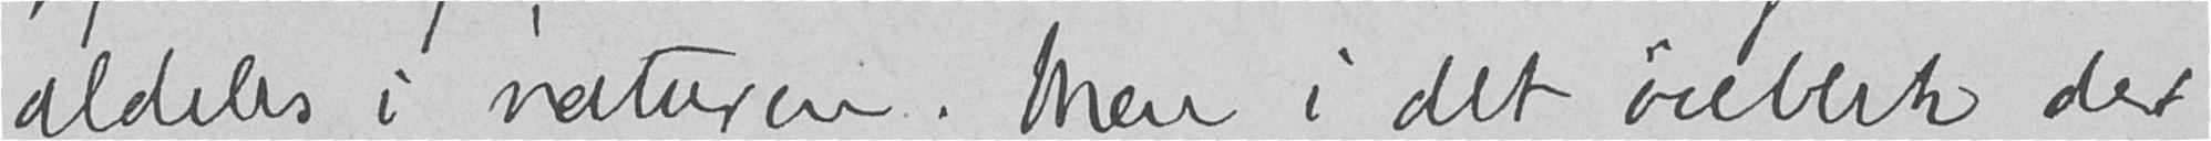aldeles i naturen. Men i det øieblik det
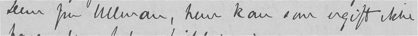Dem fru Ullman, hun kan som ugift ikke
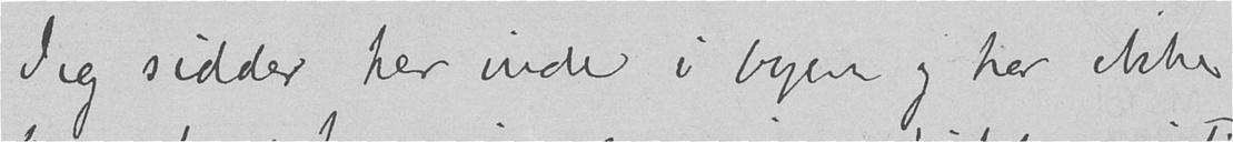Jeg sidder her inde i byen og har ikke
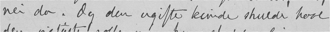nei da. Og den ugifte kvinde skulde have
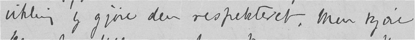vikling og gjøre den respekteret. Men kjære
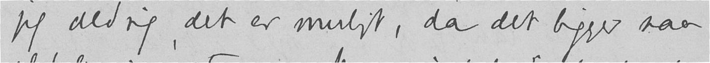jeg aldrig, det er muligt, da det ligger saa
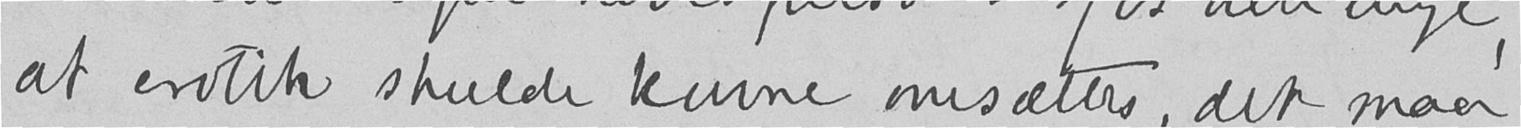at erotik skulde kunne omsættes, det maa
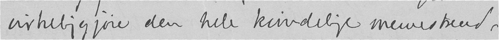virkeliggjøre den hele kvindelige menneskud-
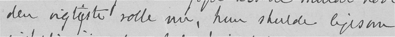den vigtigste rolle nu, hun skulde ligesom
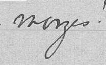morges!
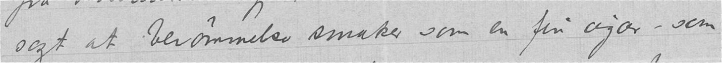sagt at berømmelse smaker som en fin cigar - som
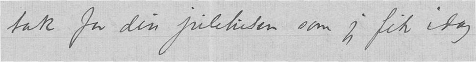tak for din julehilsen som jeg fik idag
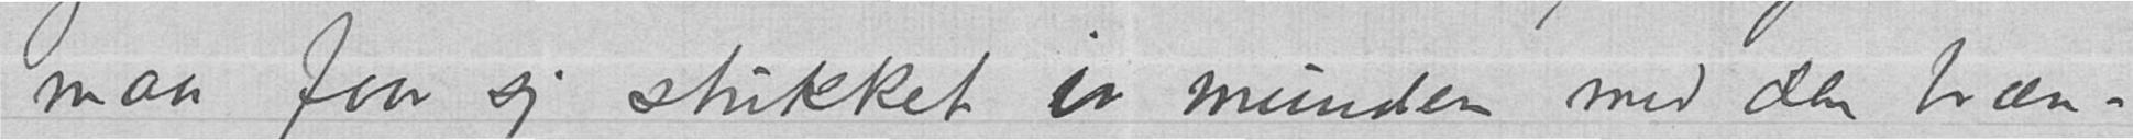man faar sig stukket i munden med den bræn-
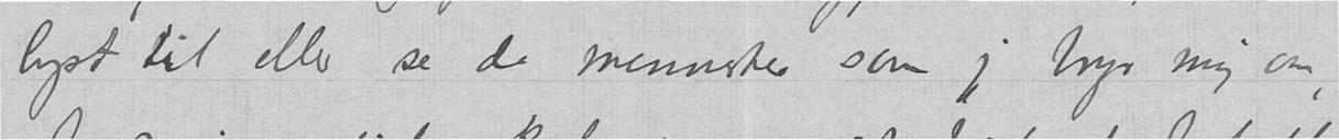lyst til eller se de mennesker som jeg bryr mig om,
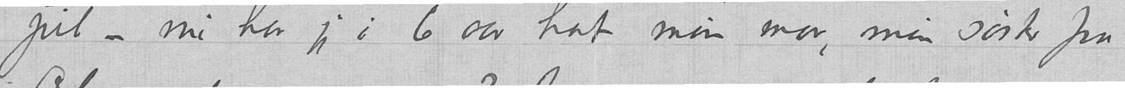jul - nu har jeg i 6 aar hat min mor, min søster fra
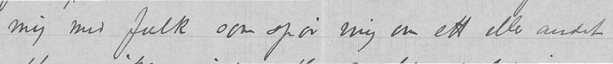mig med folk som spør mig om ett eller andet
